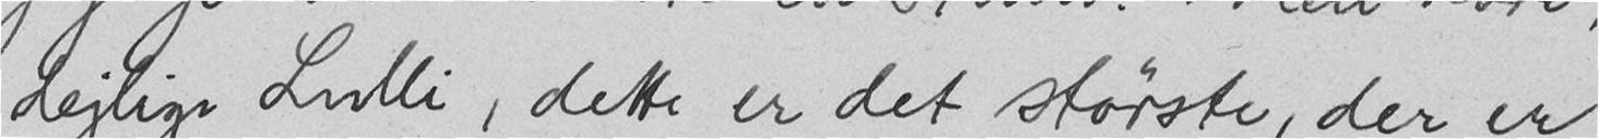dejlige Lulli, dette er det største, der er
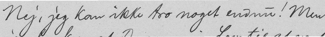Nej, jeg kan ikke tro noget endnu! Men
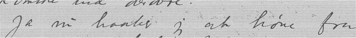Ja nu haaber jeg at høre fra
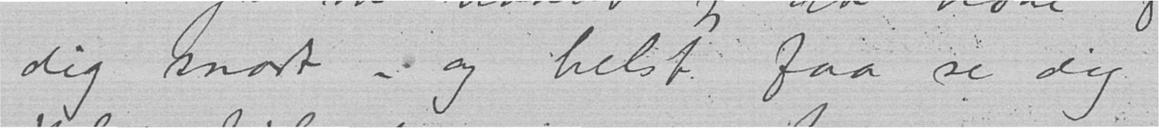dig snart - og helst faa se dig.
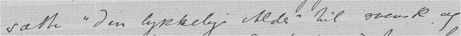sætte "Den lykkelige Alder" til svensk og
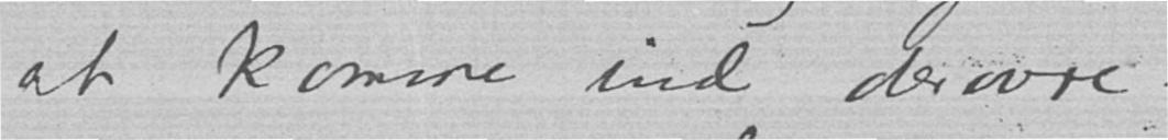at komme ind derovre.
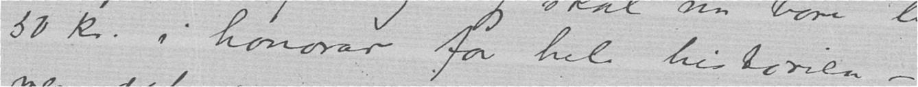50 kr. i honorar for hele historien -
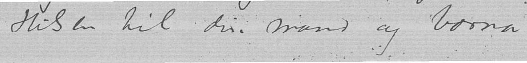Hilsen til din mand og barna
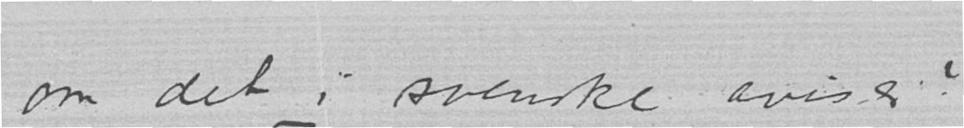om det i svenske aviser?
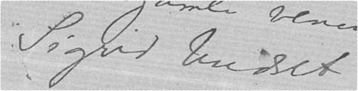Sigrid Undset.
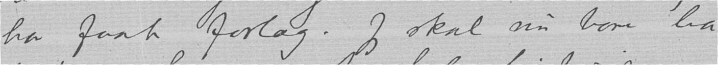har faat forlag. Jeg skal nu bare ha
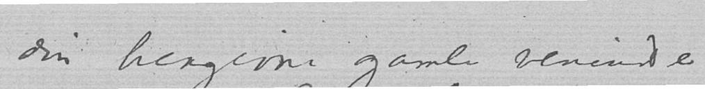din hengivne gamle veninde
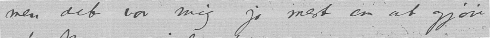men det var mig jo mest om at gjøre
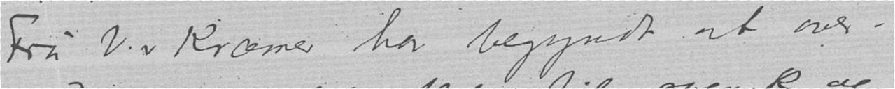Fru. V. Kræmer har begyndt at over-
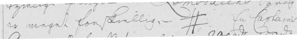er meget forskiellig. - # En Captains
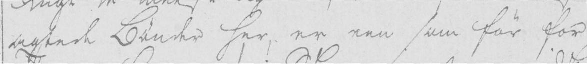agtede Bønder her, er een som før for
